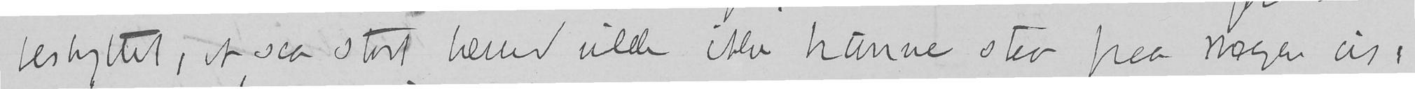beskyttet, et saa stort lerred vilde ikke kunne staa paa nogen vis,
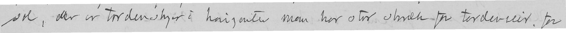sol, der er tordenskyer i horisonten man har stor skræk for tordenveir, for
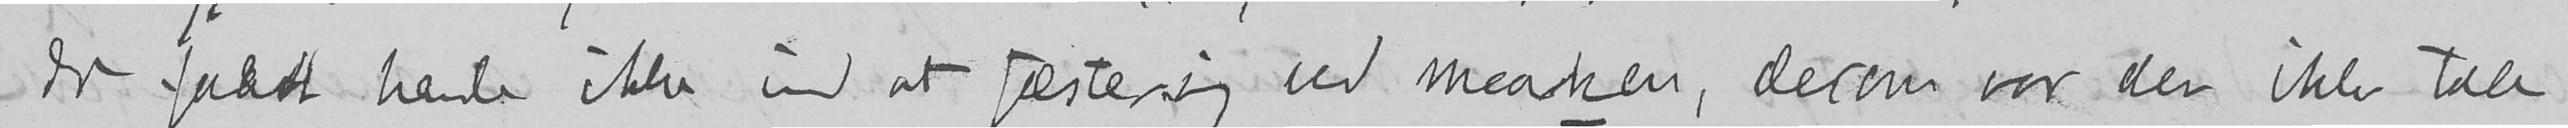det faldt hende ikke ind at fæste sig ved maaken, derom var der ikke tale,
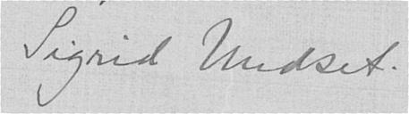Sigrid Undset.
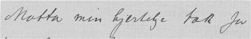Motta min hjertelige tak for
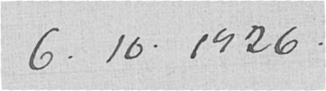6.10.1926.
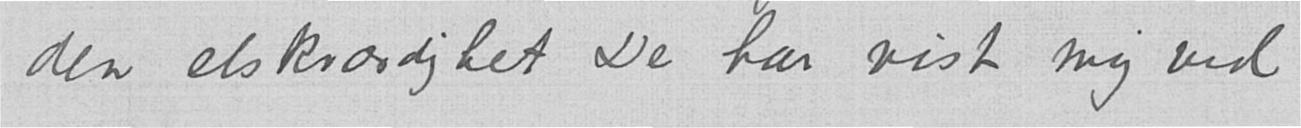den elskværdighet De har vist mig ved
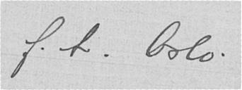f.t. Oslo.
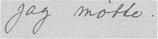jag møtte."
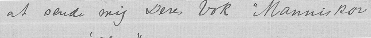at sende mig Deres bok "Människor
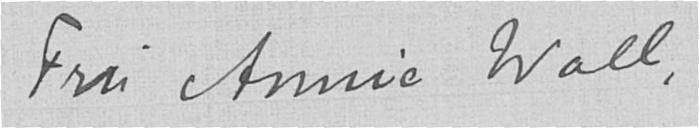Fru Annie Wall,
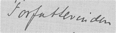Forfatterinden
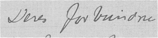Deres forbundne
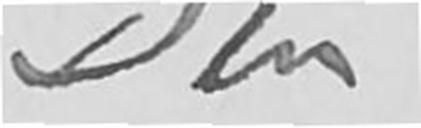Din
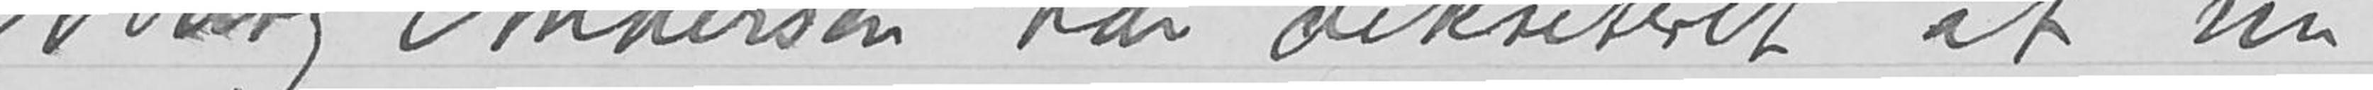Mary Andersen har dekreteret at nu
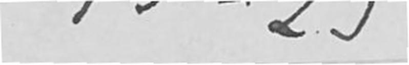20/3-23
